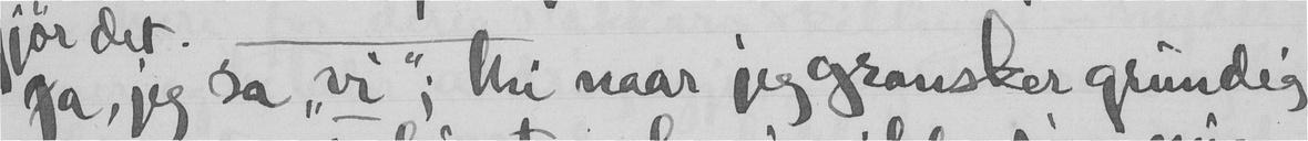Ja, jeg sa "vi"; thi naar jeg gransker grundig
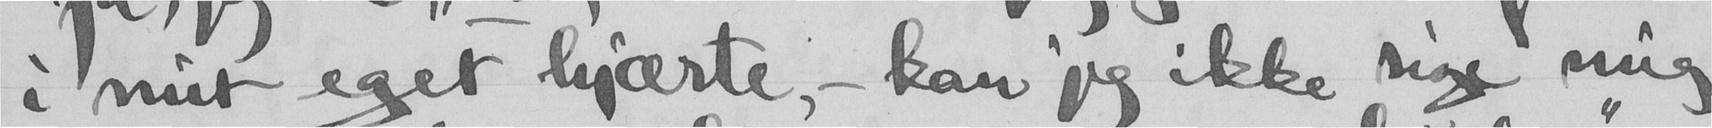i mit eget hjærte, - kan jeg ikke sige mig
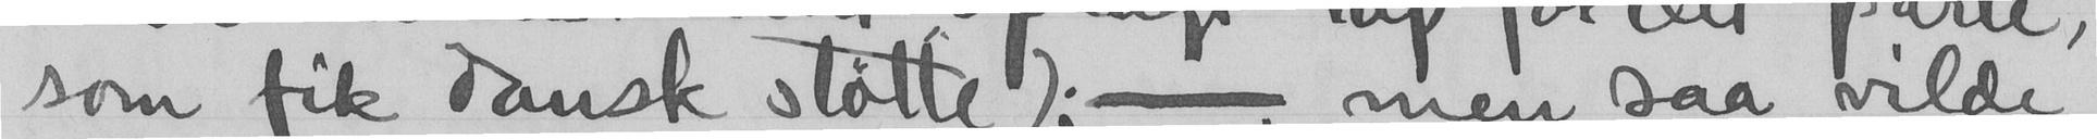som fik dansk støtte); - men saa vilde
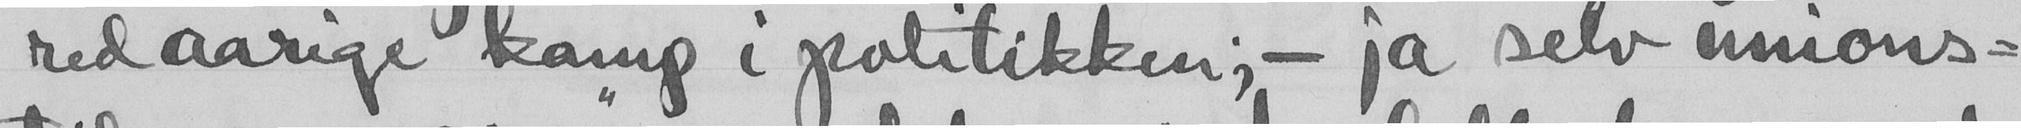red aarige kamp i politikken ; - ja selv unions-
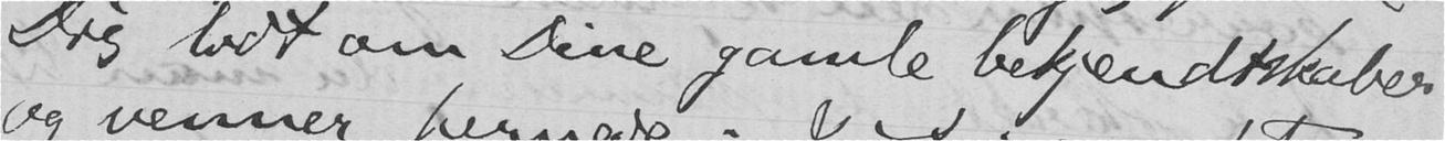Dig lidt om Dine gamle bekjendtskaber
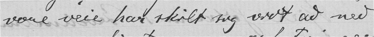vore veie har skilt sig vidt ad ned
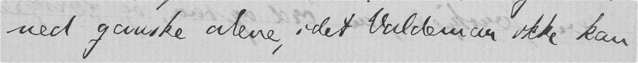ned ganske alene, idet Valdemar ikke kan
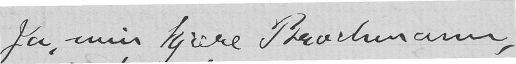Ja, min kjære Brochmann,
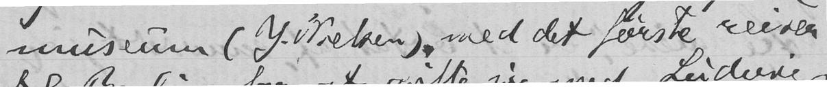museum (Y. Nielsen), med det første reiser
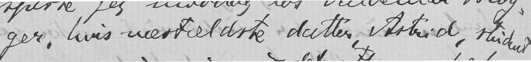ger, hvis næstældste datter, Astrid, student
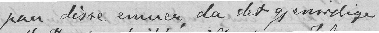paa disse emner, da det gjensidige
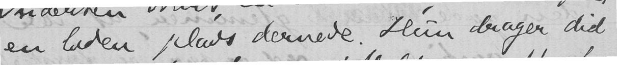en liden plads dernede. Hun drager did
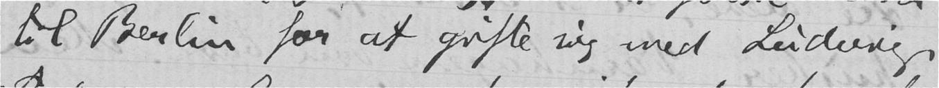til Berlin for at gifte sig med Ludvig
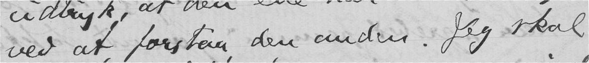ved at forstaa den anden. Jeg skal
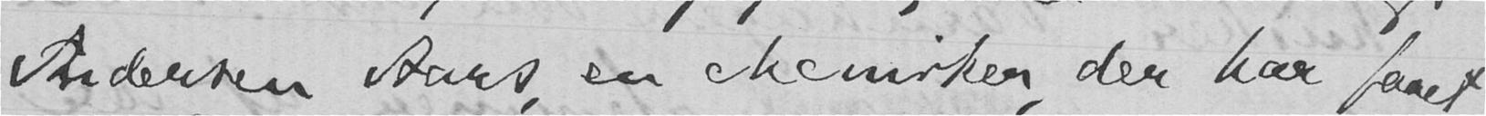Andersen Aars, en chemiker, der har faaet
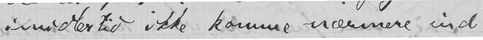imidlertid ikke komme nærmere ind
